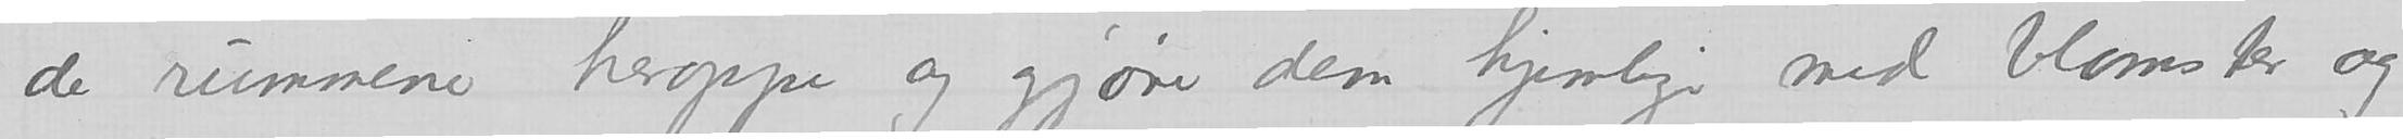de rummene heroppe og gjøre dem hjemlige med blomster og
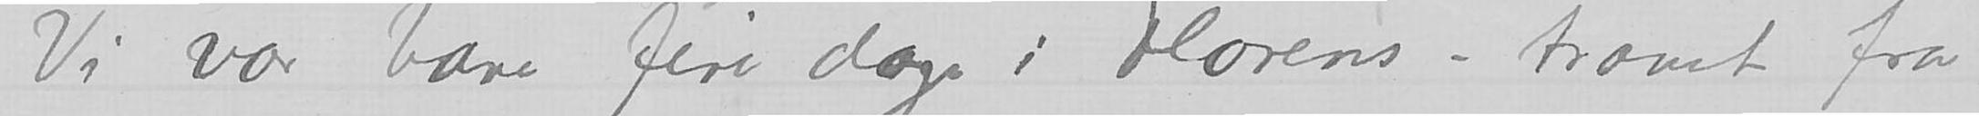Vi var bare fire dager i Florens - travet fra
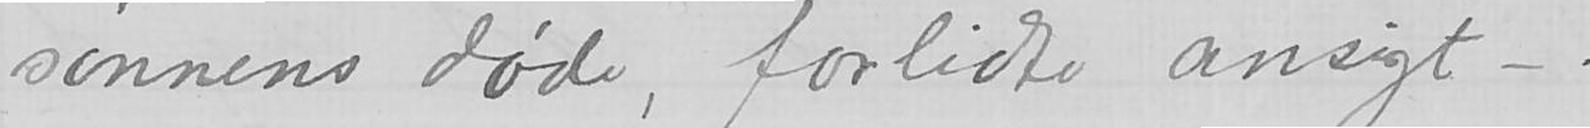sønnens døde , forlidte ansigt-
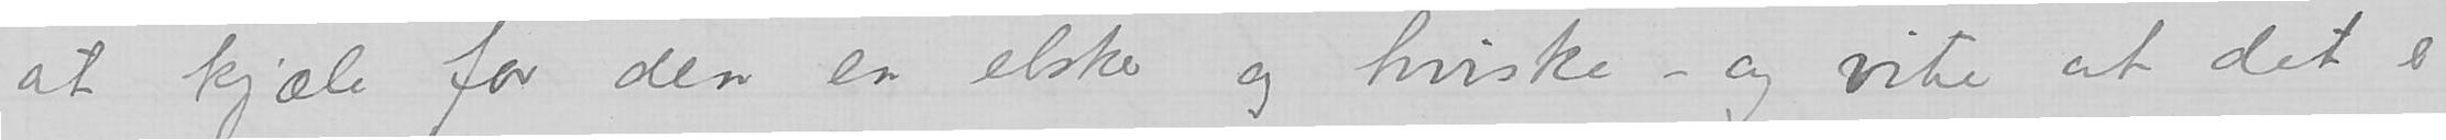at kjæle for den en elsker og hviske - og vite at det er
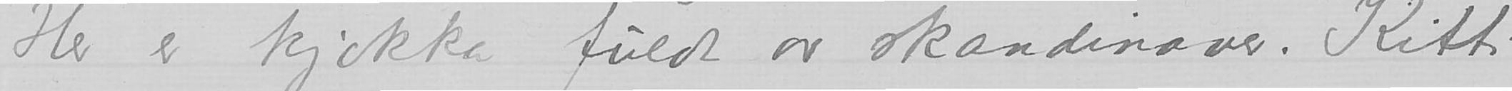Her er kjokka fuld av skandinaver. Kitti
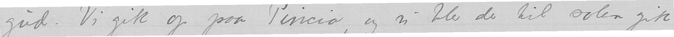gud. Vi gik op paa Pincio, og vi blev der til solen gik
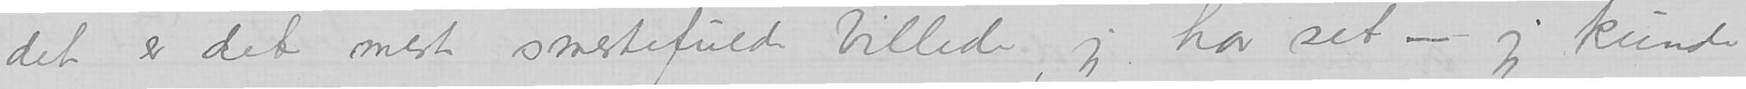-det er det mest smertefulde billede, jeg har set - jeg kunde
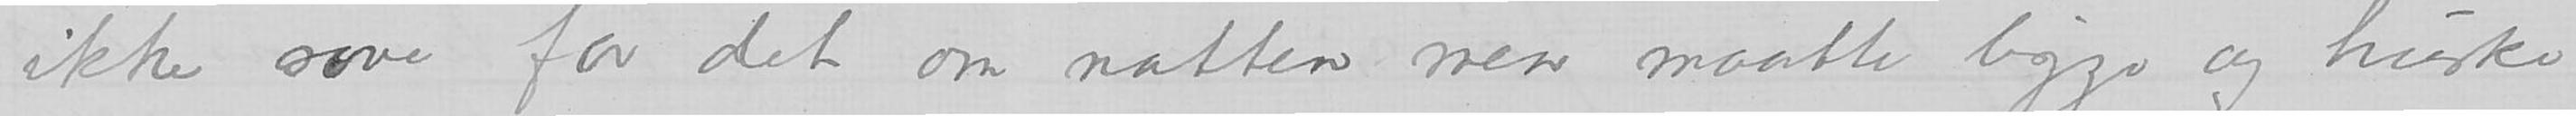ikke sove for det om natten men matte ligge og huske
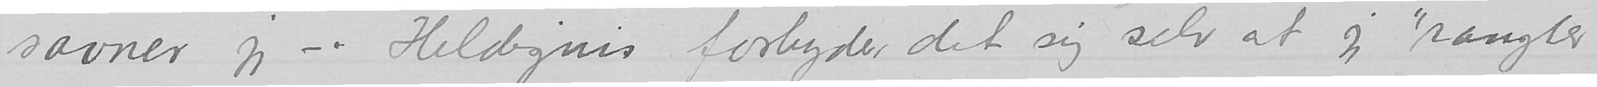savner jeg -. Heldigvis forbyder det sig selv at jeg «rangler»
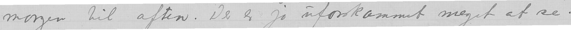morgen til aften. Der er jo uforskammet meget at se:
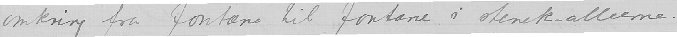omkring fra fontæne til fontæne i stenek-alleerne.
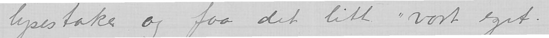og lysestaker og faa det litt «vort eget»
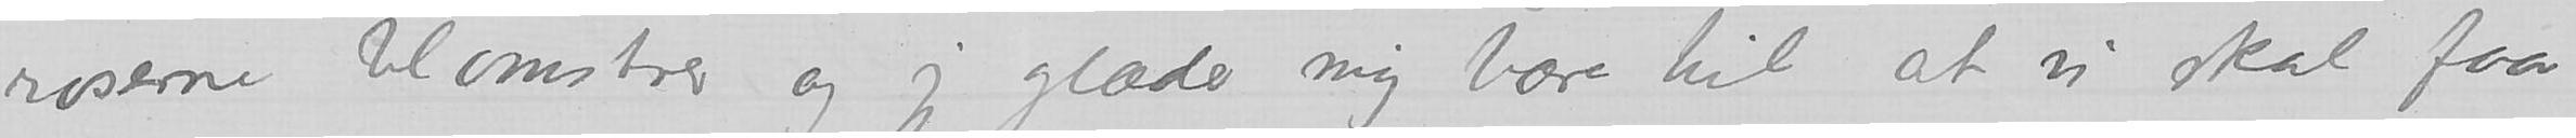roserne blomstrer og jeg glæder mig bare til at vi skal faa
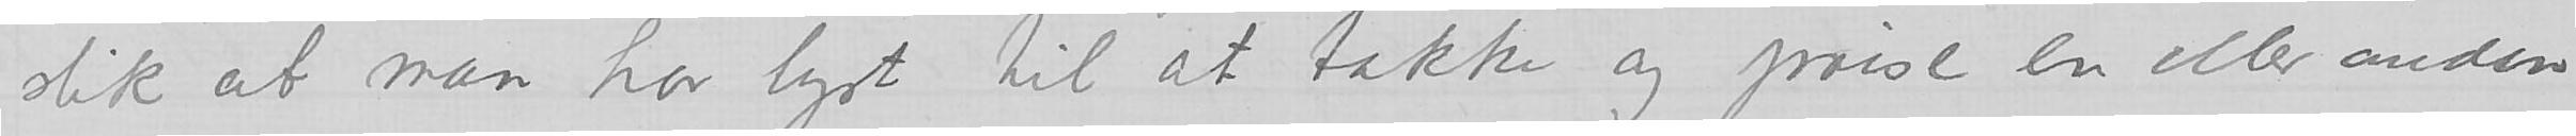slik at man har lyst til at takke og prise en eller anden
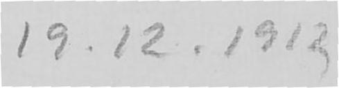19.12.1912
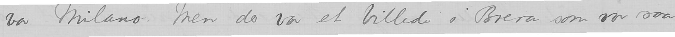var Milano. Men der var et billede i Brera som var saa –
